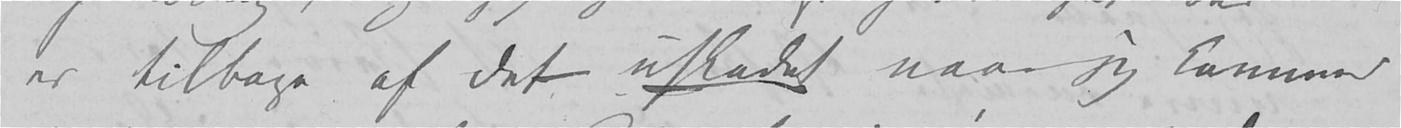tilbage af det uskadet naar jeg kommer
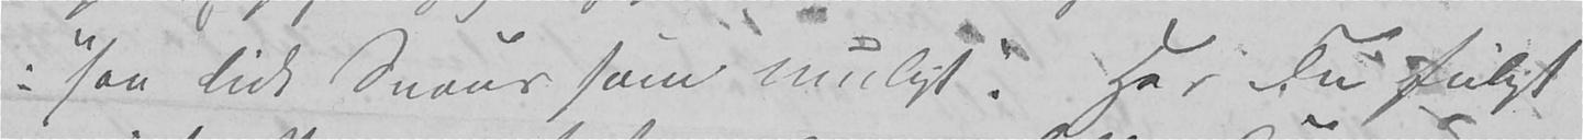«saa lidt Snaus som muligt». Har Du fulgt
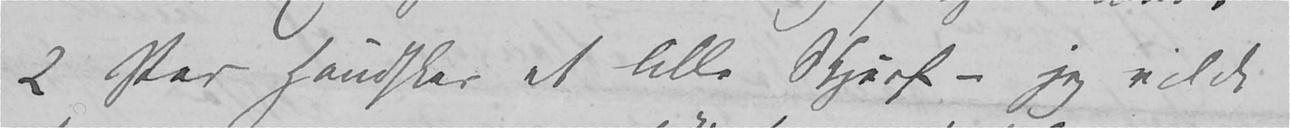2 Par Handsker et lille Skjerf – jeg vilde
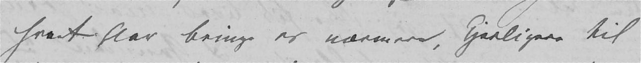hvert Aar bringe os nærmere, kjerligere til
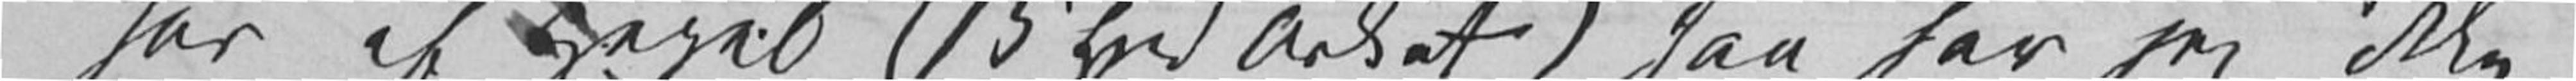har af Hegel (15 Spd Arket) saa har jeg ikke
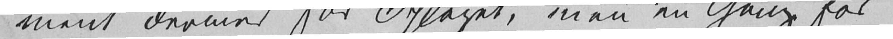ment dermed for Oplaget, men en Gang for
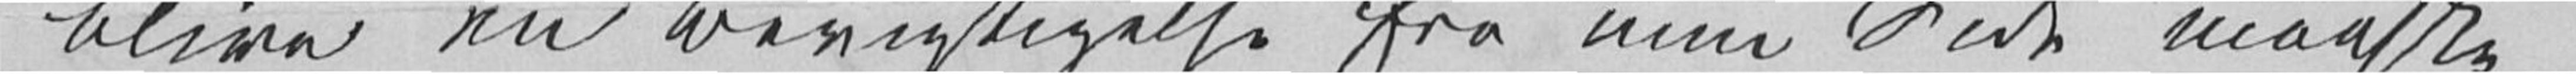blive en Berigtigelse fra min Side maaske
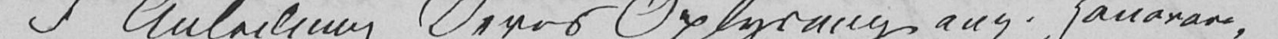I Anledning Deres Oplysning ang. Honorar,
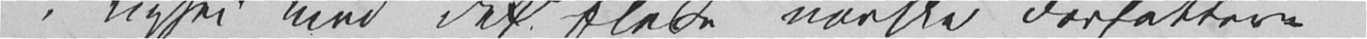i Lighed med det flere norske Forfattere
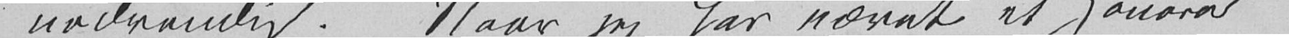nødvendig. Naar jeg har nævnt et Honorar
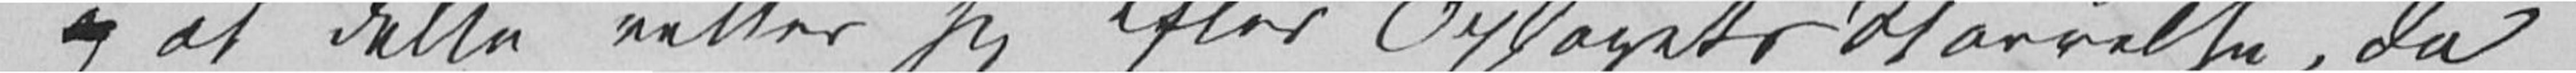og at dette retter sig efter Oplagets Størrelse, da
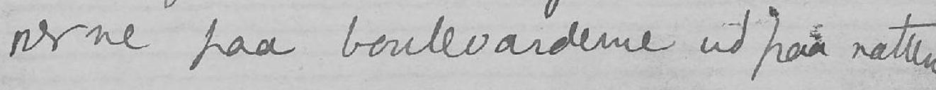serne paa boulevarderne ud paa natten
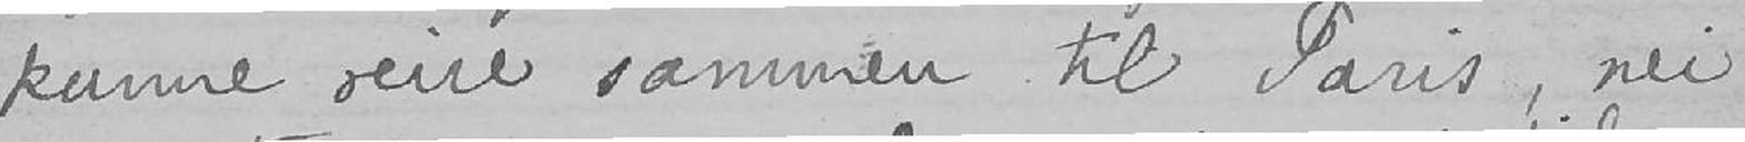kunne reise sammen til Paris, nei
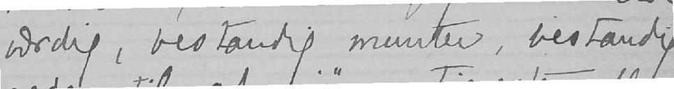værdig, bestandig munter, bestandig
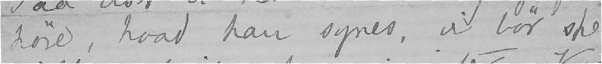høre, hvad han synes, vi bør spe-
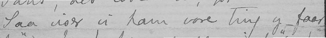Saa viser vi ham vore ting og faar
